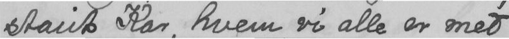staut Kar, hvem vi alle er med
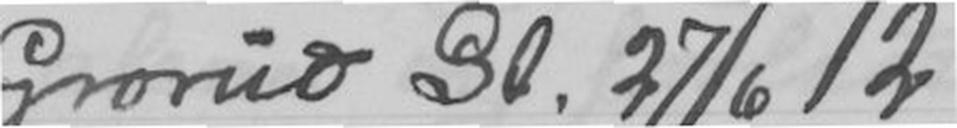Grorud St. 27/6 12
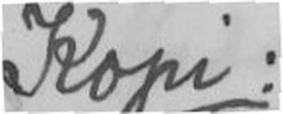Kopi:
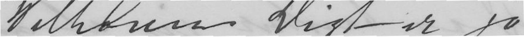Welhavens Digt er jo
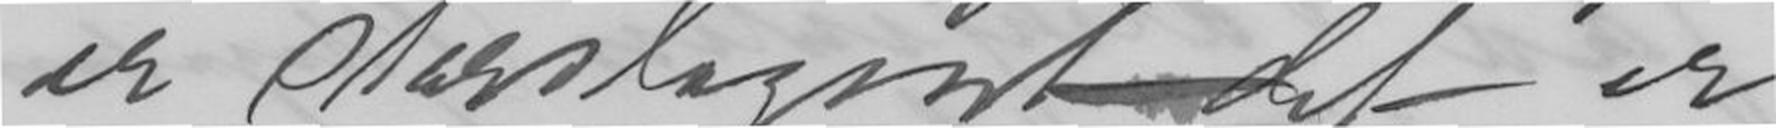er storslagent det er
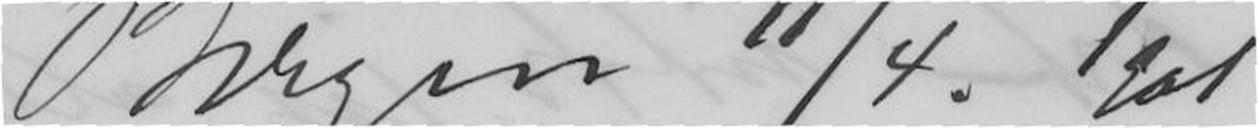Bergen 11/4. 1901
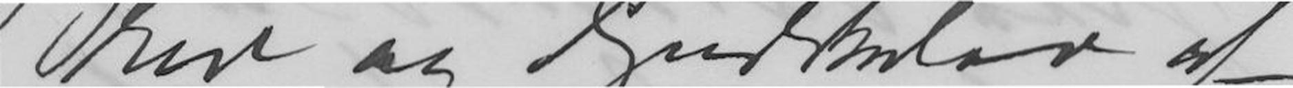Brev og Gudskelov at
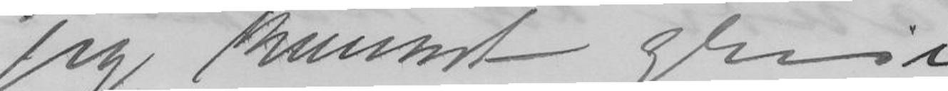jeg kunnet greie
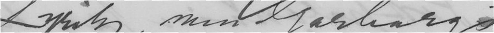Lyrik, men Garborgs
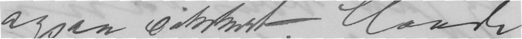ogsaa sikkert. Havde
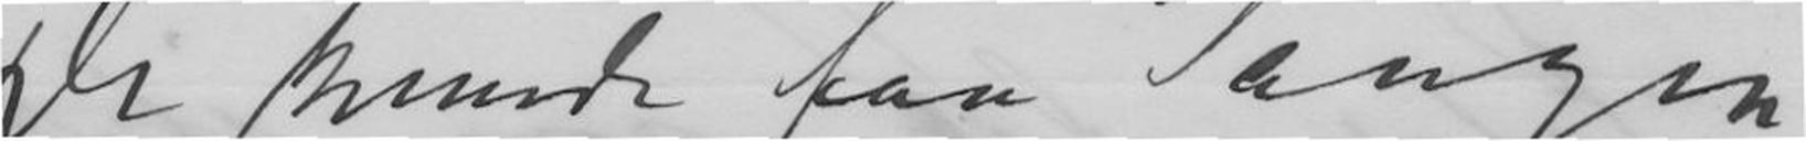De kunde faa Sangen
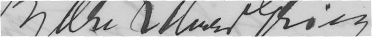Kjære Edvard Grieg
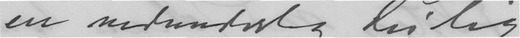en vidunderlig deilig
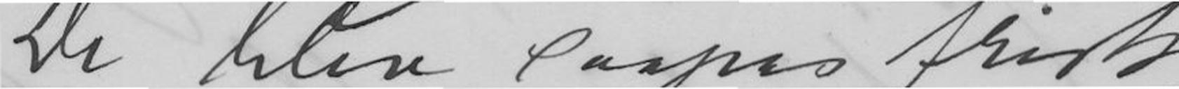De blev saapas frisk
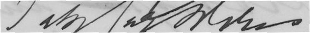Tak for Deres
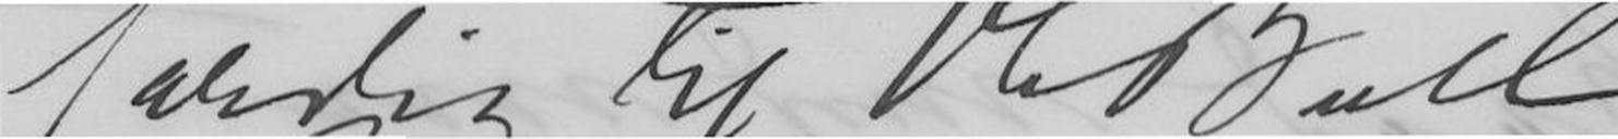færdig til Ole Bull.
